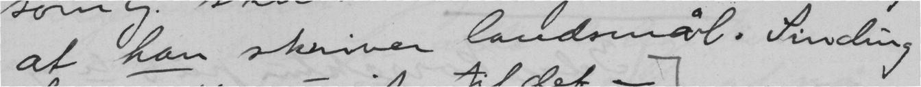at han skriver landsmål. Sinding
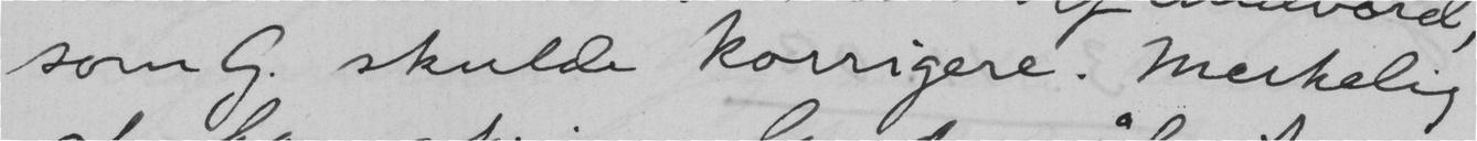som G. skulde korrigere. Merkelig
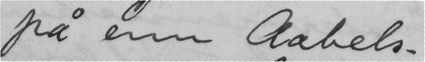på enn Aabels.
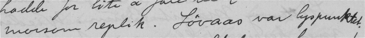morsom replik. Løvaas var lyspunktet;
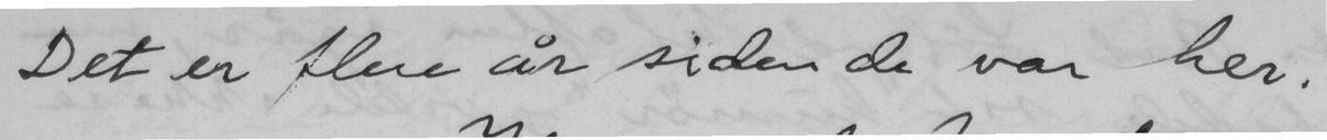Det er flere år siden de var her.
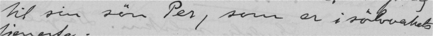til sin søn Per, som er i sølvverkets
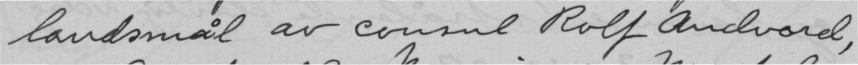landsmål av consul Rolf Andvord,
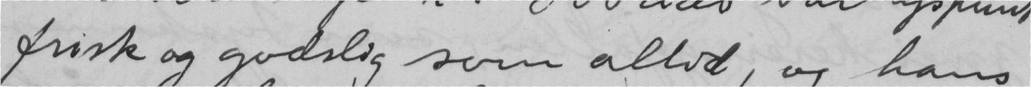frisk og godslig som altid, og hans
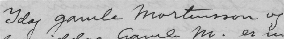Idag gamle Mortensson og
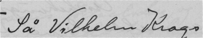Så Vilhelm Krags
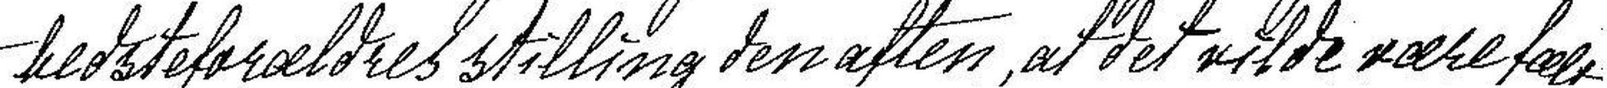bedsteforældres stilling den aften, at det vilde være fælt
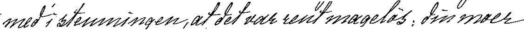med i stemningen, at det var mageløs; din moer
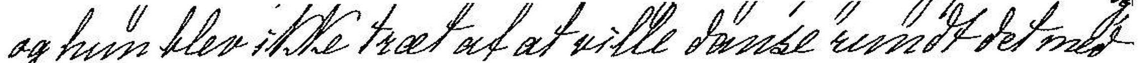og hun blev ikke træt af at ville danse rundt det med
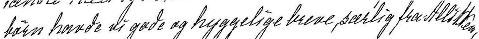børn havde vi gode og hyggelige brev, særlig Allikken,
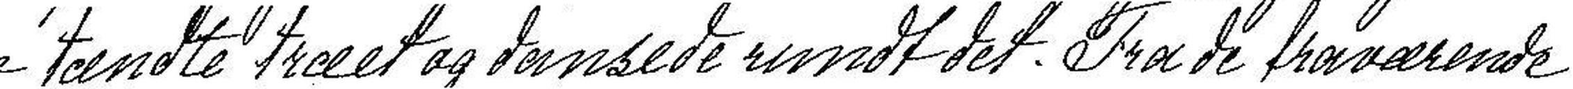tænte træet og dansede rundt det. Fra de fraværende
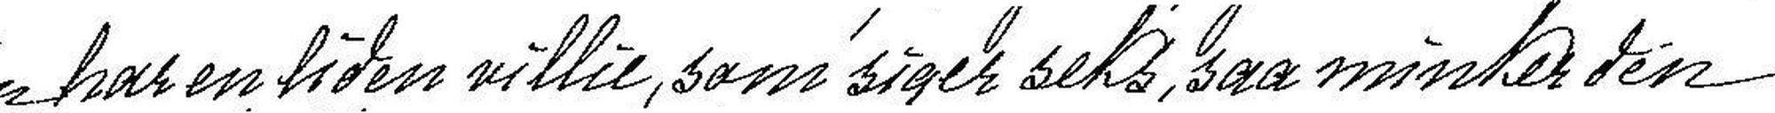har en liden villie, som siger seks, saa minker den
